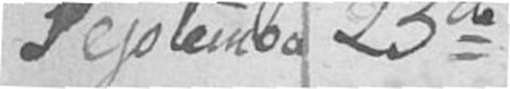September 23de
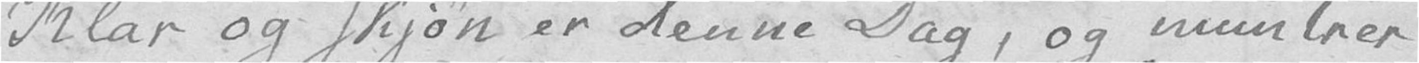Klar og skjøn er denne Dag, og muntrer
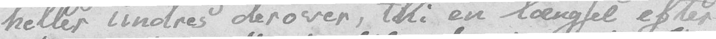heller undres derover, thie en længsel efter
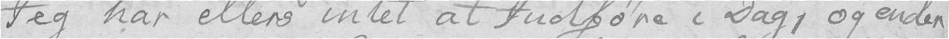Jeg har ellers intet at Indføre i Dag, og ender
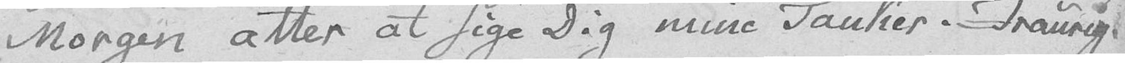Morgen atter at sige Dig mine Tanker. – Traurig.
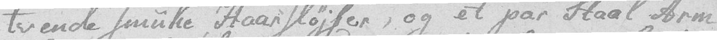tvende smuke Haarsløjfer, og et par Staal Arm
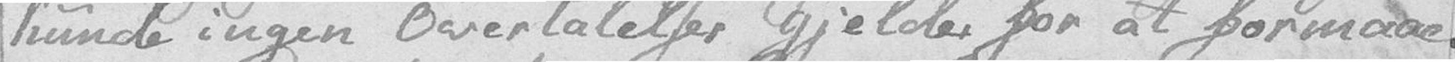kunde ingen Overtalelser gjelde, for at formaae
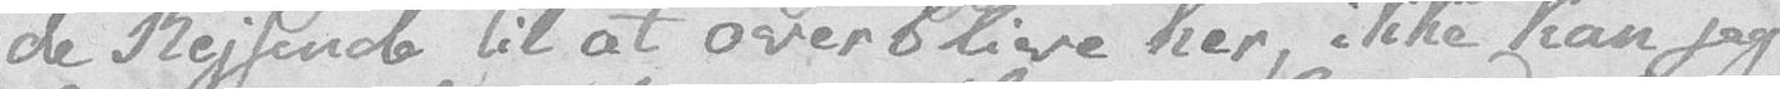de Rejsende til at overblive her, ikke kan jeg
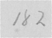182
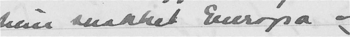hun snakket Europa og
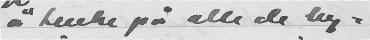å tenke på alle de bry-
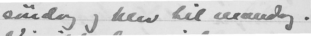søndag og blev til mandag.
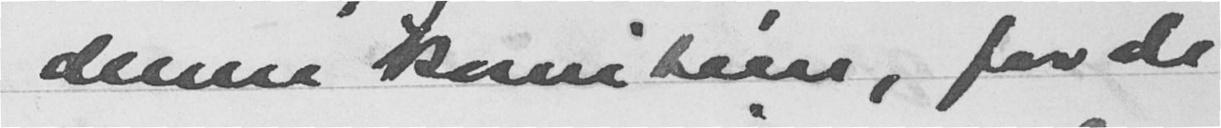denne komiteen, fordi
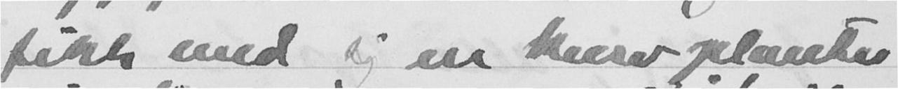fikk med sig en kurvplante
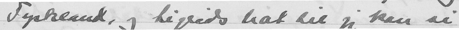Tykland, og Sigrids hat til jeg kan si
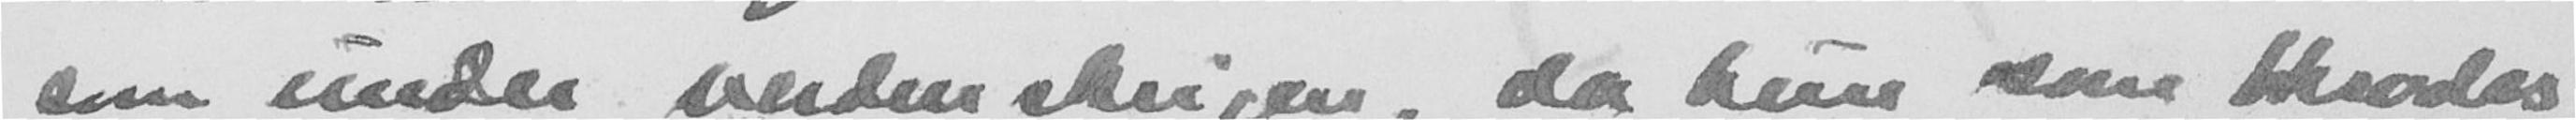som under verdenskrigen, da hun som trvdes
